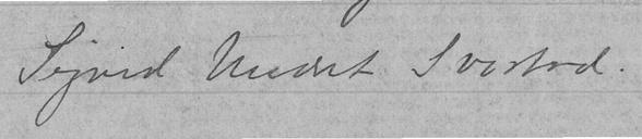Sigrid Undset Svarstad.
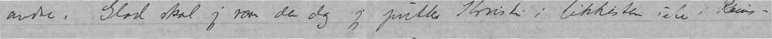andre. Glad skal jeg være den dag jeg putter Kristin i likkisten ute i Reins-
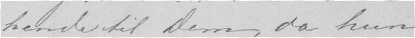hende til Dem, da hun
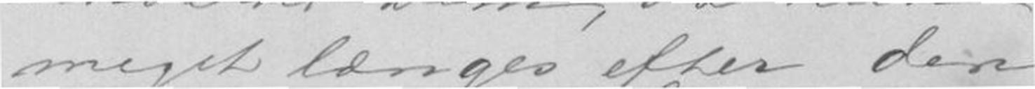meget længes efter den
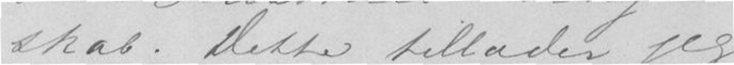skab. Dette tillader jeg
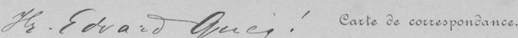Hr. Edvard Grieg!
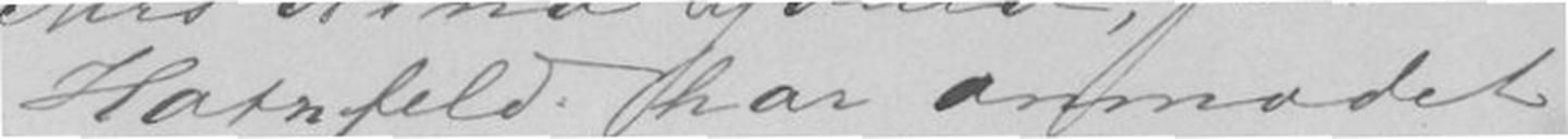Hatnfeld har anmodet
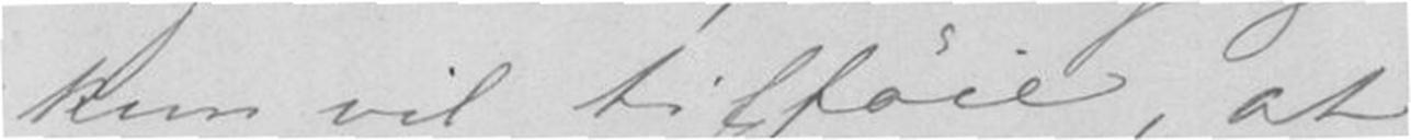kun vil tilføie, at
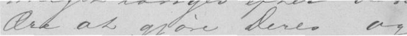Ære at gjøre Deres og
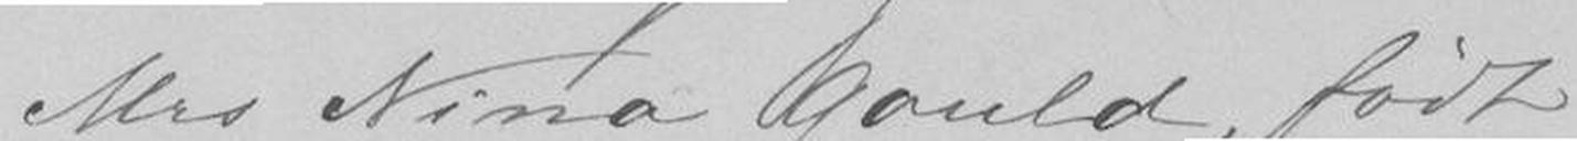Mrs Nina Gould, født
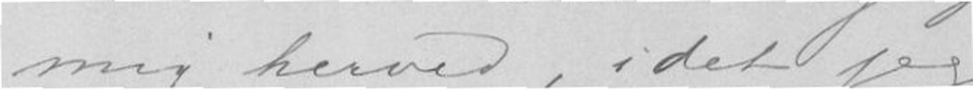mig herved, idet jeg
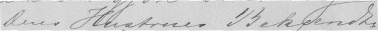Deres Hustrus Bekjendts
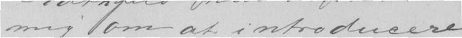mig om at introducere
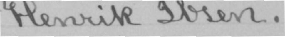Henrik Ibsen.
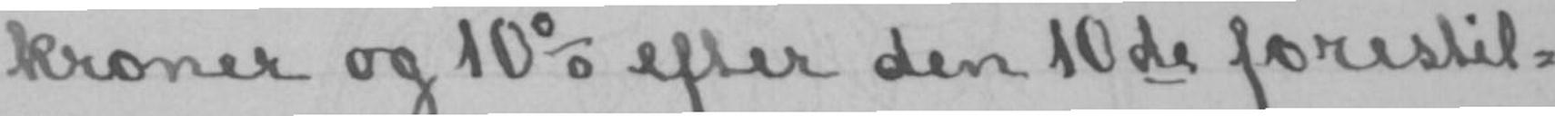kroner og 10 % efter den 10de forestil-
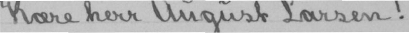Kære herr August Larsen!
